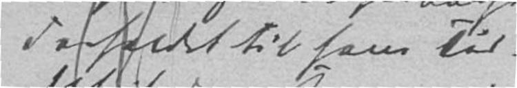Forholdet til hans Tid.
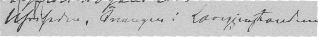Ufriheden, Tvangen i Læregjenstandene
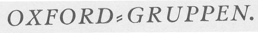OXFORD-GRUPPEN.
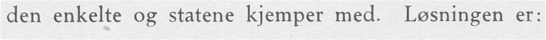den enkelte og statene kjemper med. Løsningen er:
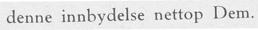denne innbydelse nettop Dem.
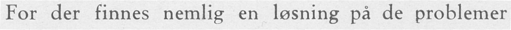For der finnes nemlig en løsning på de problemer
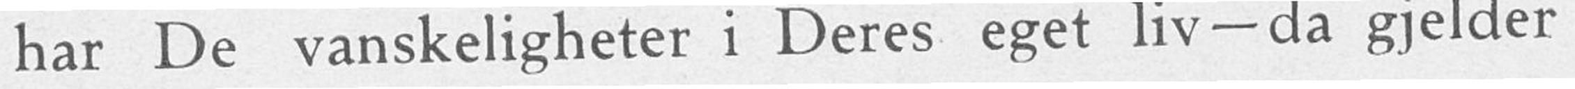har De vanskeligheter i Deres eget liv - da gjelder
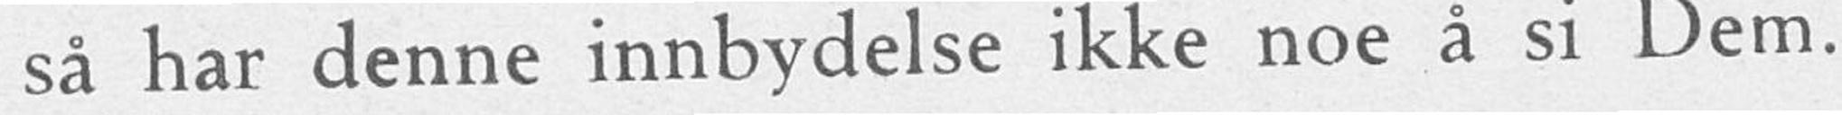så har denne innbydelse ikke noe å si Dem.
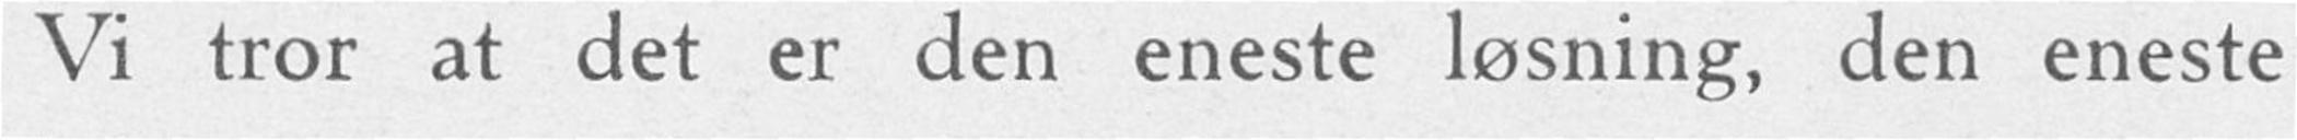Vi tror at det er den eneste løsning, den eneste
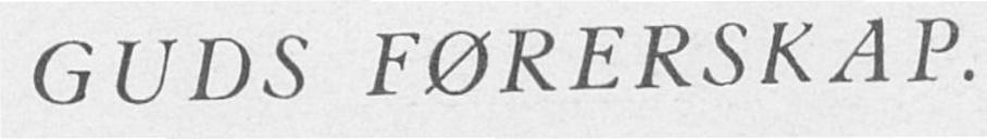GUDS FØRERSKAP.
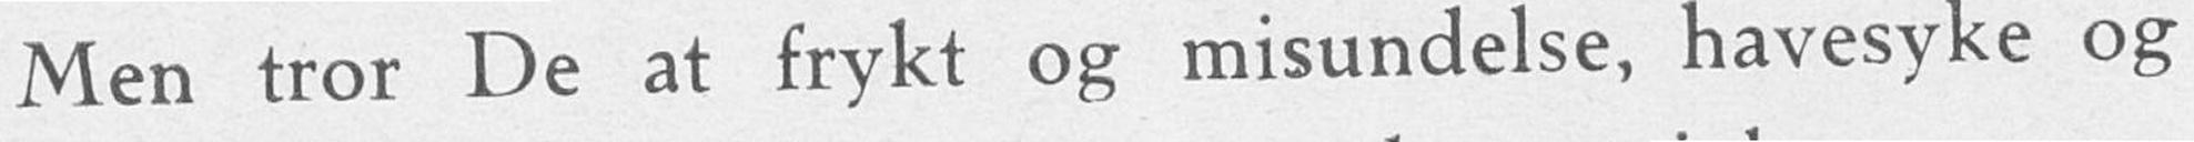Men tror De at frykt og misundelse, havesyke og
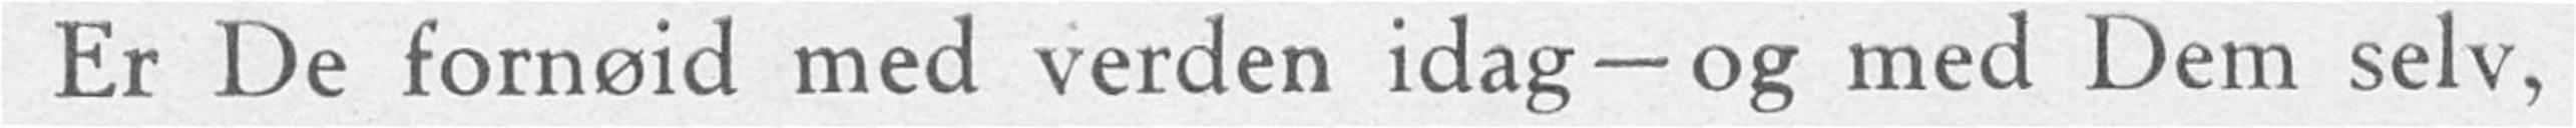Er De fornøid med verden idag — og med Dem selv,
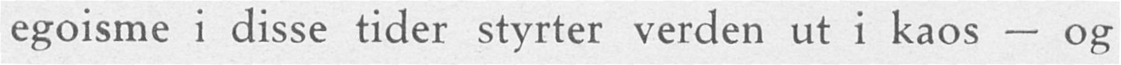egoisme i disse tider styrter verden ut i kaos - og
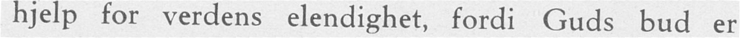hjelp for verdens elendighet, fordi Guds bud er
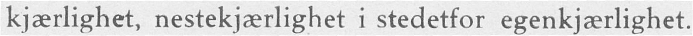kjærlighet, nestekjærlighet i stedetfor egenkjærlighet.
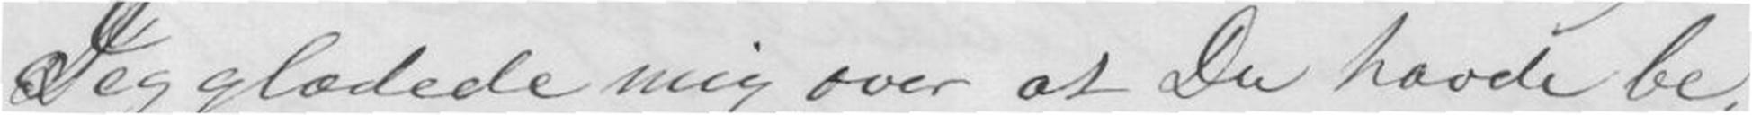Jeg glædede mig over at Du havde be-
